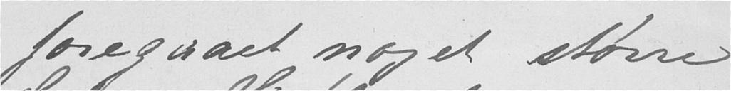foregaaet noget større
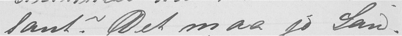lant? Det maa jo Sand-
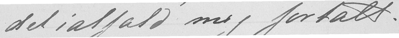det ialfald mig fortalt.
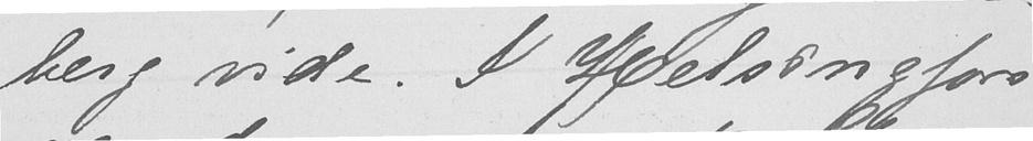berg vide. I Helsingfors
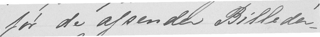før de afsender Billeder-
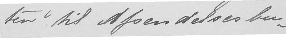ten til Afsendelsesbu-
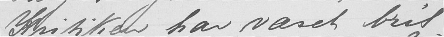Kritiken har været bril-
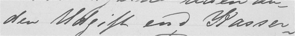den Udgift end Kasser-
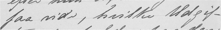faa vide, hvilke Udgif-
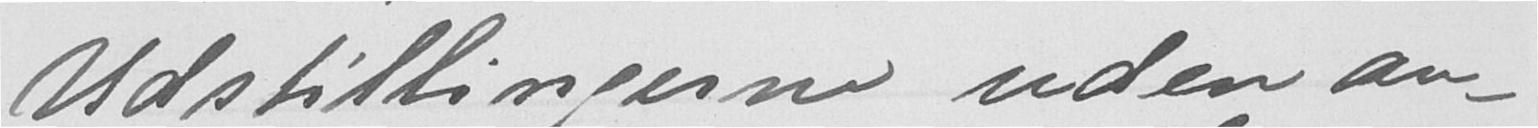Udstillingerne uden an-
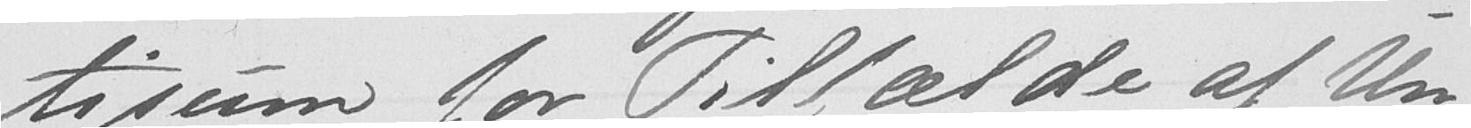tisum for Tilfælde af Un-
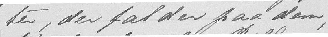ter, der falder paa dem,
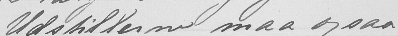Udstillerne maa ogsaa
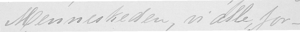Menneskeden, vi alle, for-
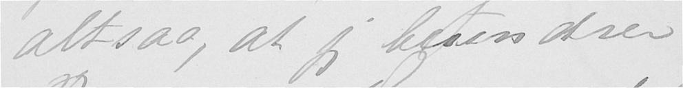altsaa, at jeg beundrer
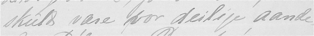skulde være vor deilige aande-
